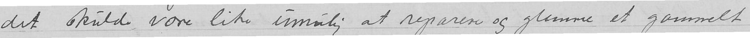det skulde være like umulig at reparere og glemme et gammelt
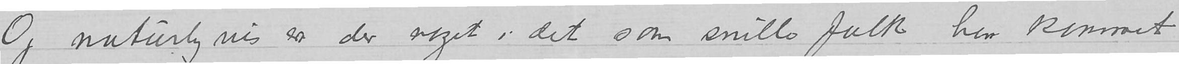Og naturligvis er der noget i det som snille folk har kommet
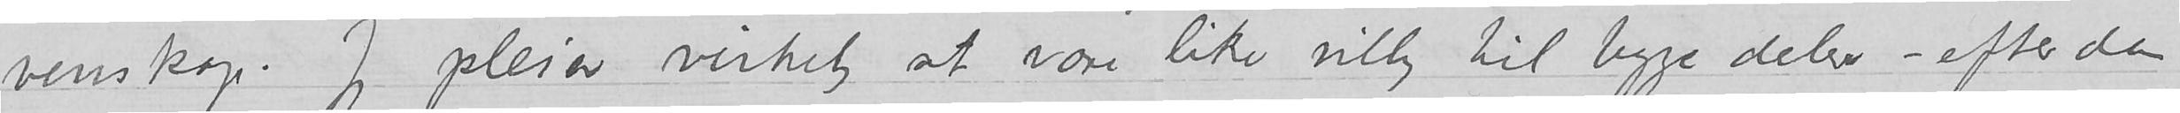venskap. Jeg pleier virkelig at være like villig til begge deler - efter den
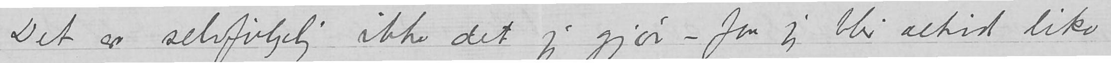Det er selvfølgelig ikke det jeg gjør - for jeg blir altid like
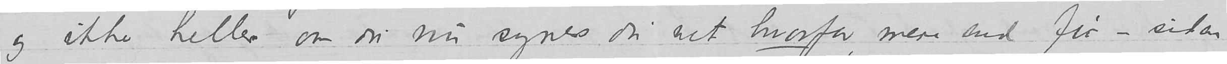og ikke heller om du nu synes du vet hvorfor, mere end før -
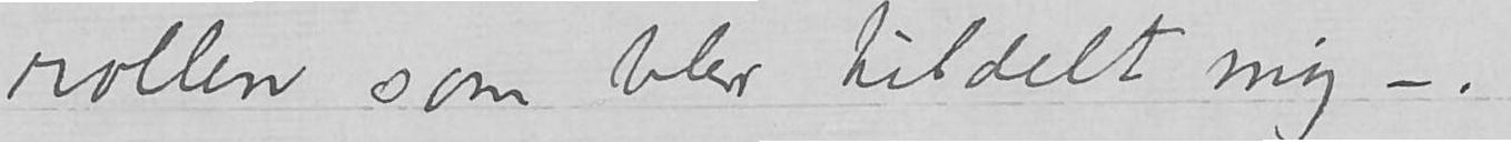rollen som blev tildelt mig -.
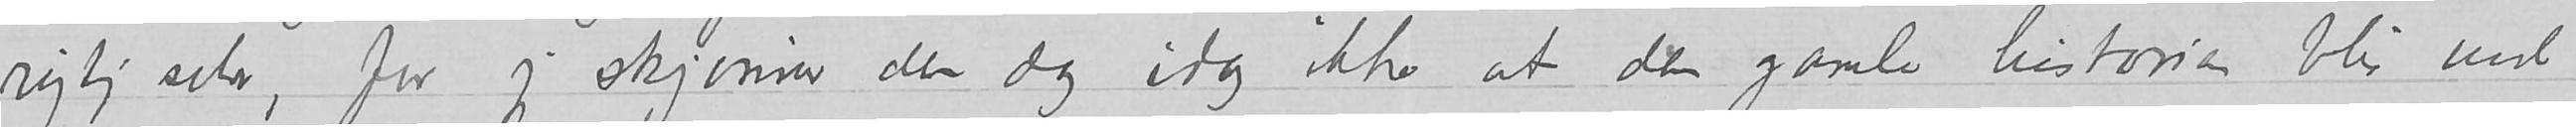rigtig selv, for jeg skjønner den dag idag ikke at den gamle historien blir ved
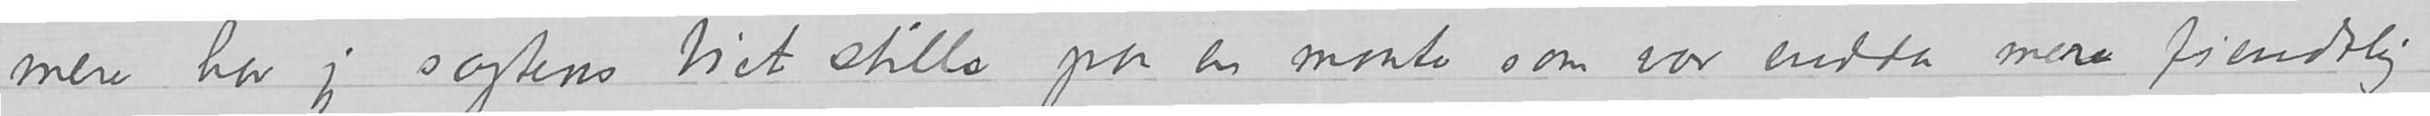mere har jeg sagtens tiet stille paa en maate som var endda mere fiendtlig -
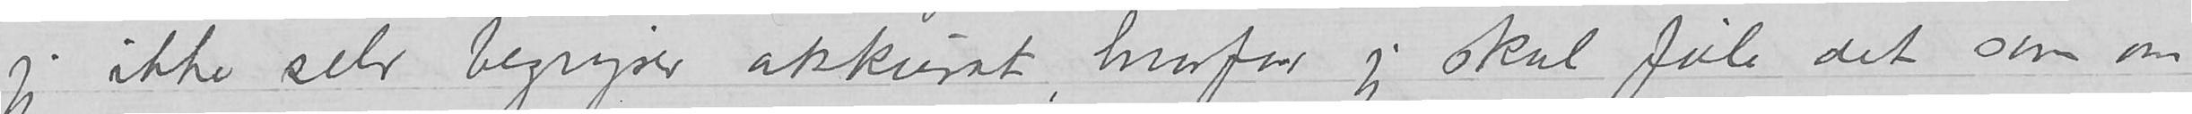siden jeg ikke selv begriper akkurat, hvorfor jeg skal føle det som om
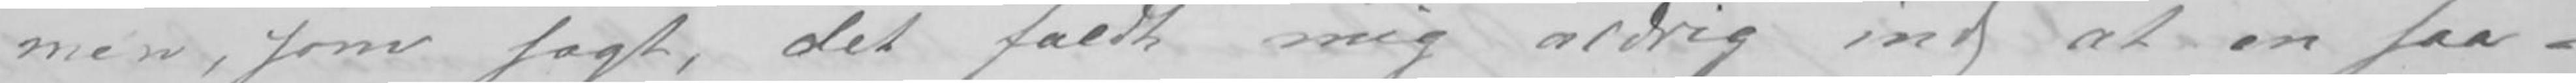men, som sagt, det faldt mig aldrig ind at en saa-
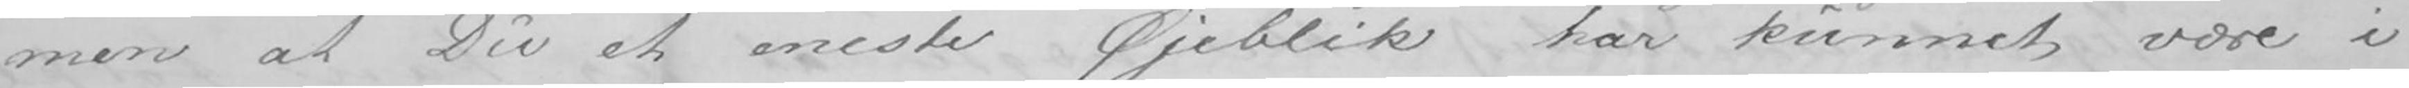men at Du et eneste Øjeblik har kunnet være i
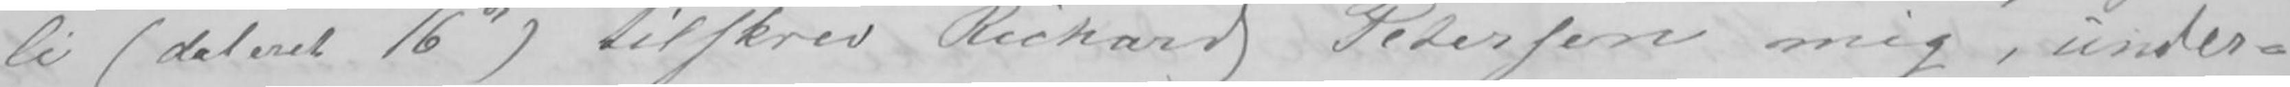li (dateret 16de) tilskrev Richard Petersen mig, under-
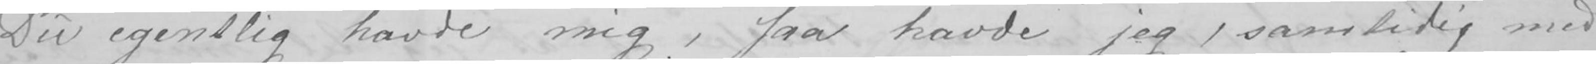Du egentlig havde mig, saa havde jeg, samtidig med
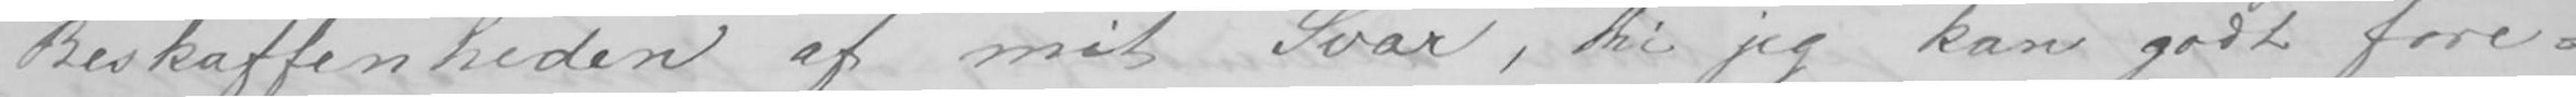Beskaffenheden af mit Svar, thi jeg kan godt fore-
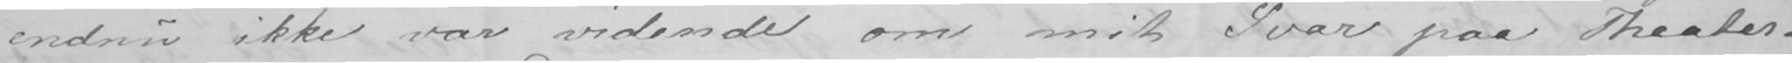endnu ikke var vidende om mit Svar paa Theater-
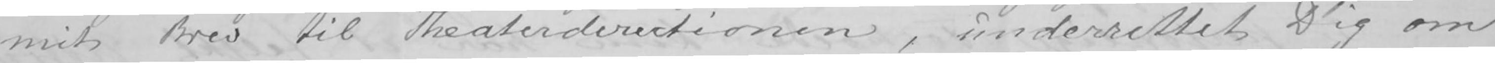mit Brev til Theaterdirectionen, underrettet Dig om
